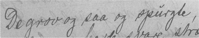De grov og saa og spurgte,
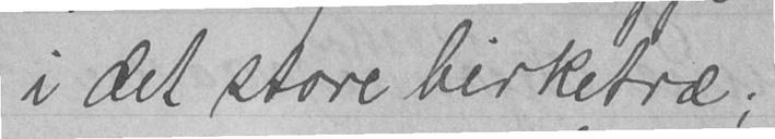i det store birketræ;
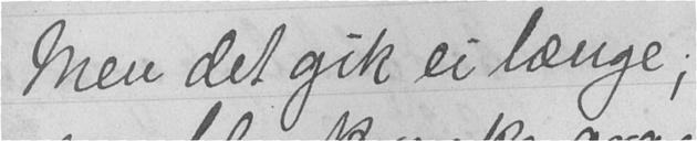Men det gik ei længe;
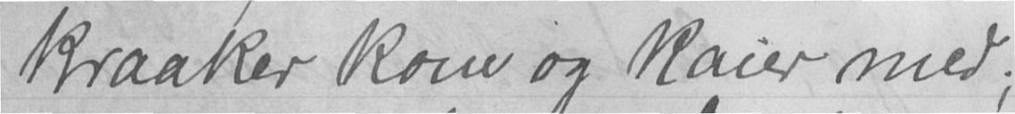kraaker kom og kaier med;
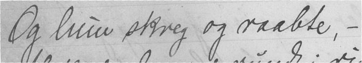Og hun skreg og raabte, -
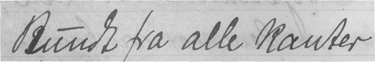Bundt fra alle kanter
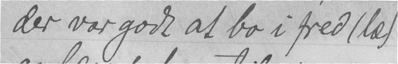der var godt at bo i fred (læ)
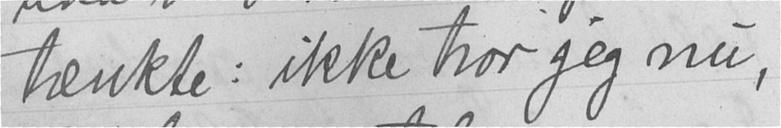tenkte: ikke tror jeg nu,
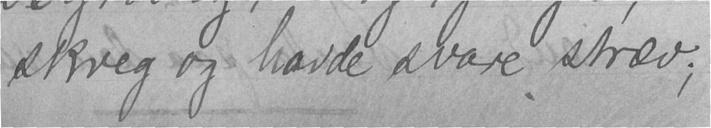skveg og havde svare stræv;
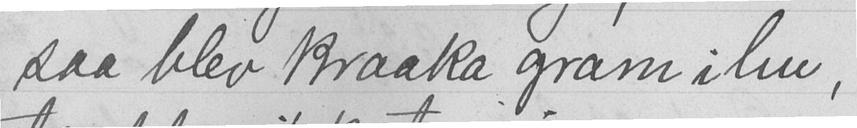saa blev kraaka gram i hu,
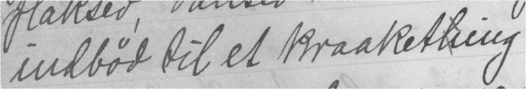indbød til et kraakething
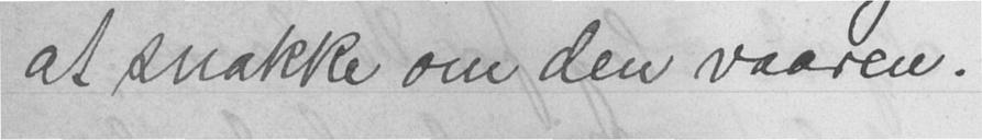at snakke om den vaaren.
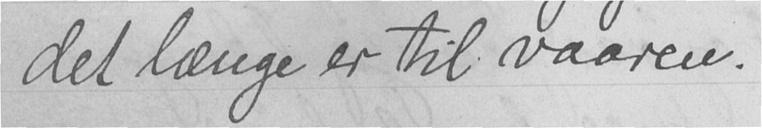det længe er til vaaren.
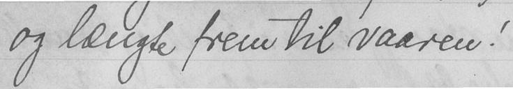og længte frem til vaaren!
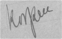korpen
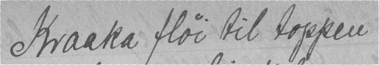Kraaka fløi til toppen
